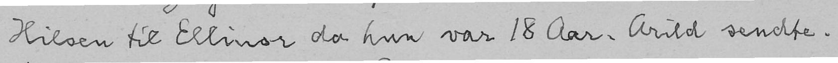Hilsen til Ellinor da hun var 18 Aar. Arild sendte.
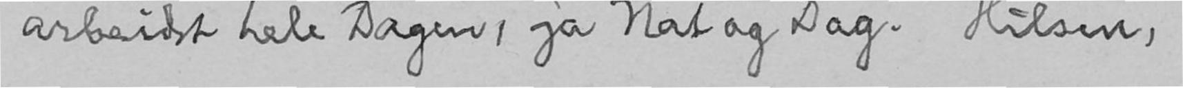arbeidet hele Dagen, ja Nat og Dag. Hilsen,
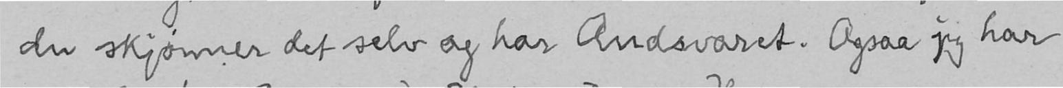du skjønner det selv og har Andsvaret. Ogsaa jeg har
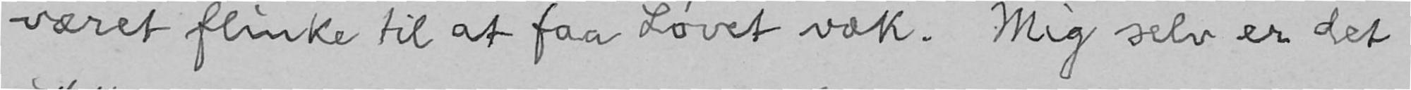været flinke til at faa Løvet væk. Mig selv er det
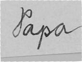Papa
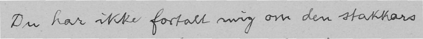Du har ikke fortalt mig om den stakkars
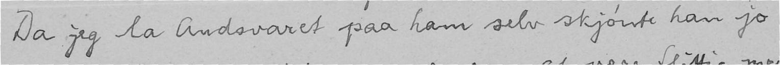Da jeg la Andsvaret paa ham selv skjønte han jo
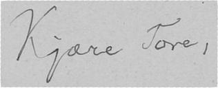Kjære Tore,
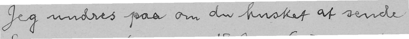Jeg undres paa om du husket at sende
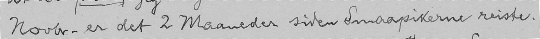Novbr. er det 2 Maaneder siden Smaapikerne reiste.
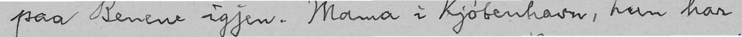paa Benene igjen. Mama i Kjøbenhavn, hun har
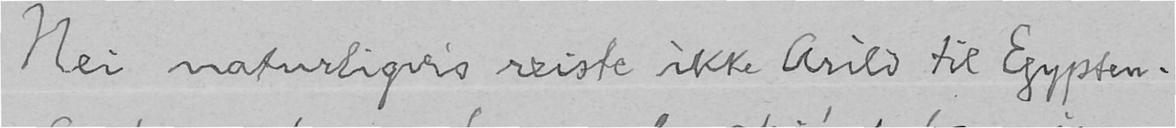Nei naturligvis reiste ikke Arild til Egypten.
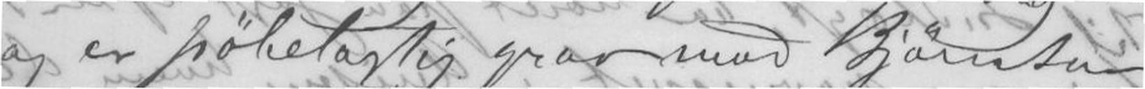og er pøbelagtig grov mod Bjørnson
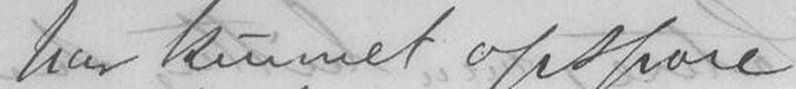har kunnet opspore
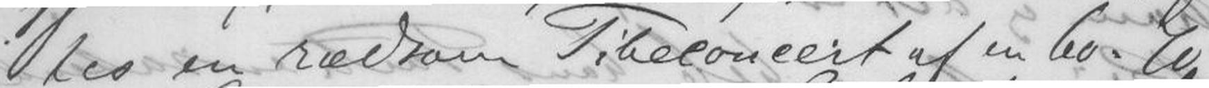tes en rædsom Pibeconcert af en 60-70
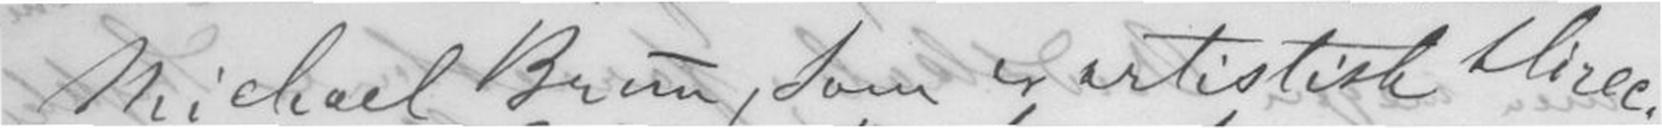Michael Brun, som er artistisk Direc-
